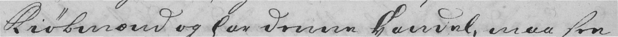Kiøbmænd og har denne Handel, maa see
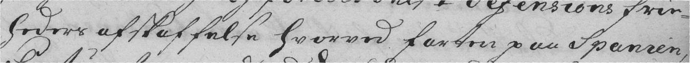heders afskaffelse hvorved farten paa Spanien,
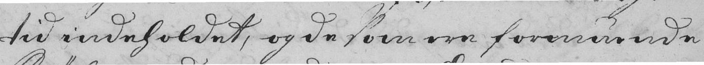tid indeholdet, og de som ere formuende
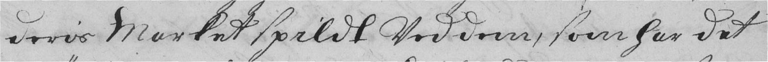deris Market spildt Ved dem, som har det
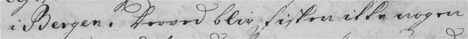i Bergen. Derved blir Fisken ikke nogen
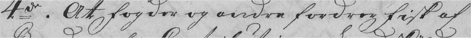4de. At Fogder og andre fordrer Fisk af
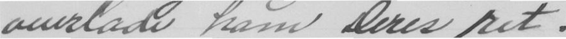overlade ham Deres ret.
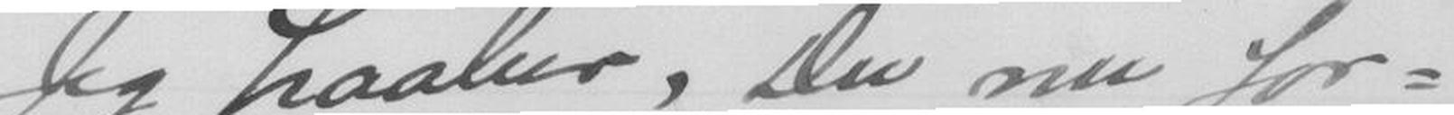Jeg haaber, Du nu for-
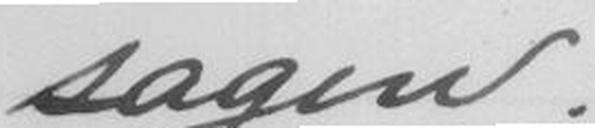sagen.
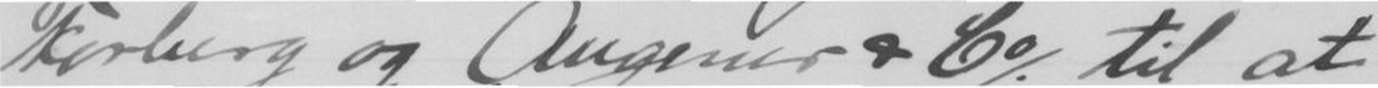Forberg og Augener & Co. til at
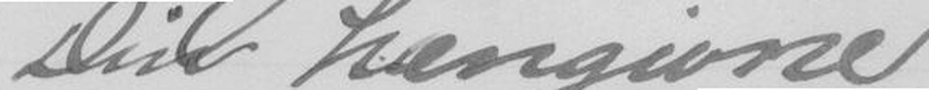Din hængivne
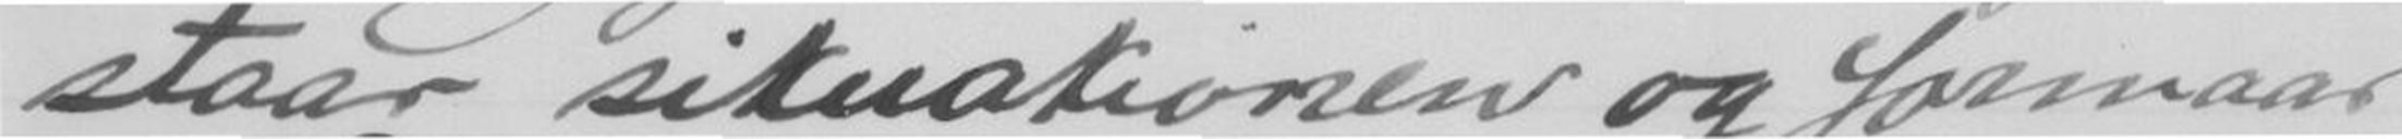staar situationen og fornaar
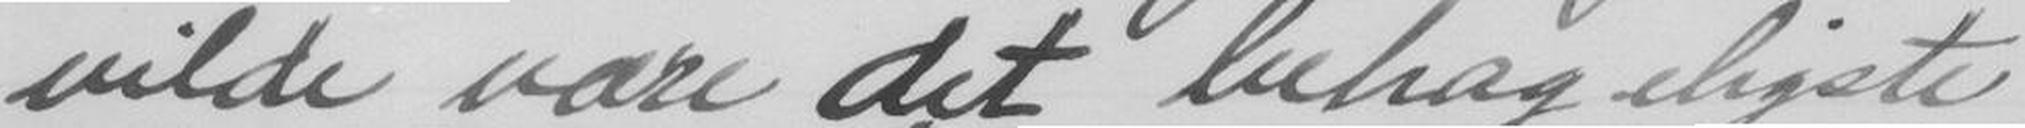vilde være det behageligste,
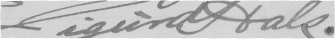Sigrud Hals.
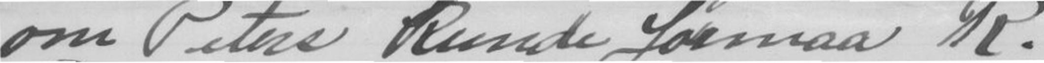om Peters kunde formaa R.
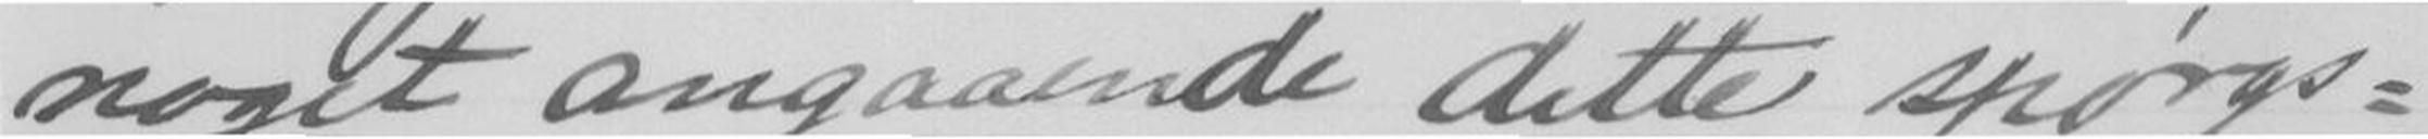noget angaaende dette spørgs-
